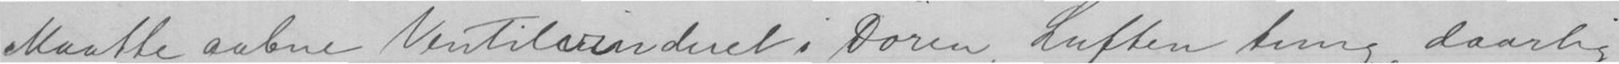Maatte aabne Ventilvinduet i Døren, Luften tung, daarlig
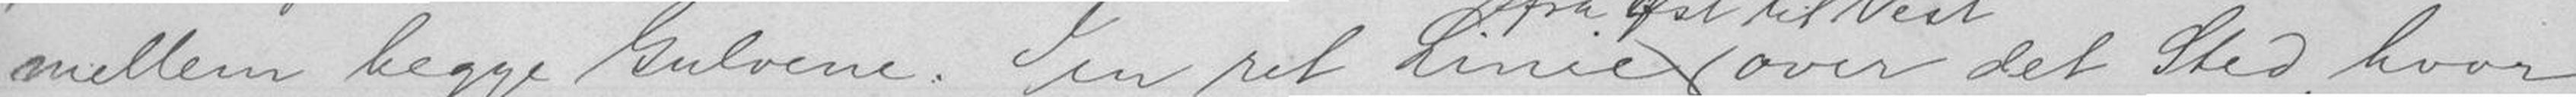mellem begge Gulvene. I en ret Linie over det Sted, hvor
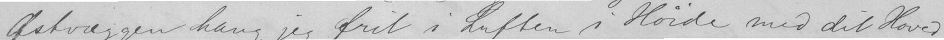Østvæggen hang jeg frit i Luften i Høide med dit Hoved
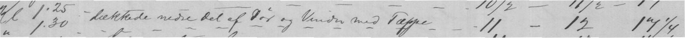" 1.30 _ _ _ _ _ _ _ _ _ _ _ _ _ _ _ _ _ _ _ _ 11 - 12 - 17¼
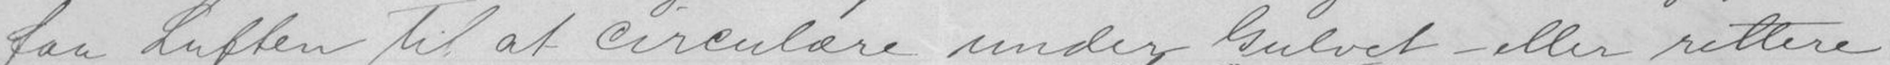faa Luften til at circulere under Gulvet - eller rettere
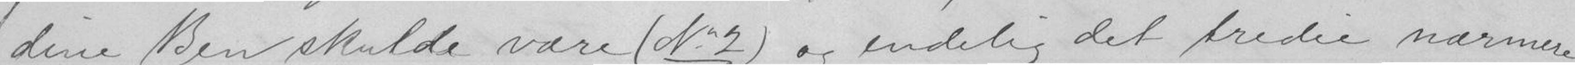dine Ben skulde være (N.2) og endelig det tredie nærmere
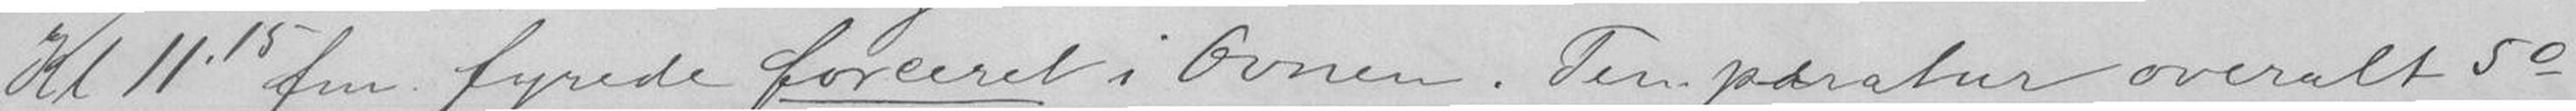Kl 11.15 fm. fyrede forceret i Ovnen. Temperatur overalt 5.
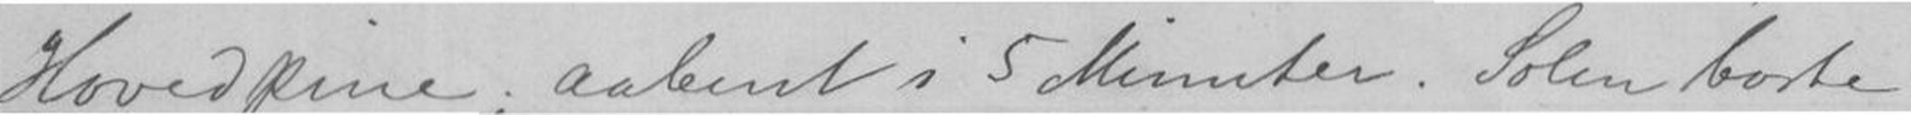Hovedpine; aabent i 5 Minuter. Solen borte
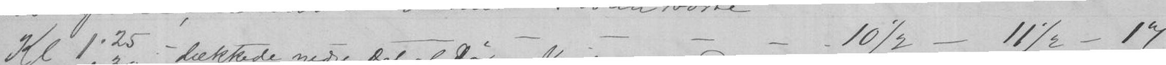Kl 1.25 dækkede nedre del af Dør og Vindu med Tæppe 10½ - 11½ - 17
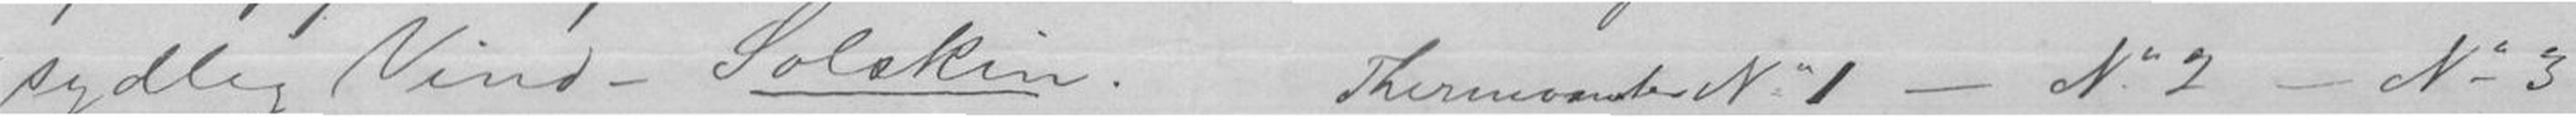sydlig Vind - Solskin. ThermometerN.1 - N.2 - N.3
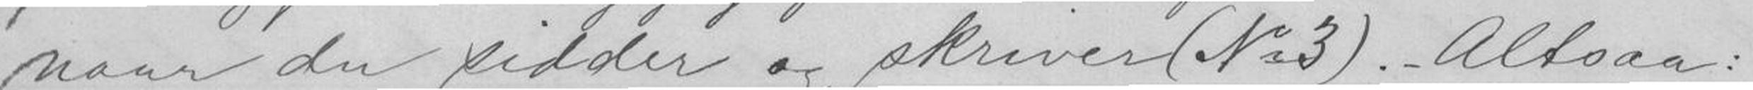naar du sidder og skriver (N.3). - Altsaa:
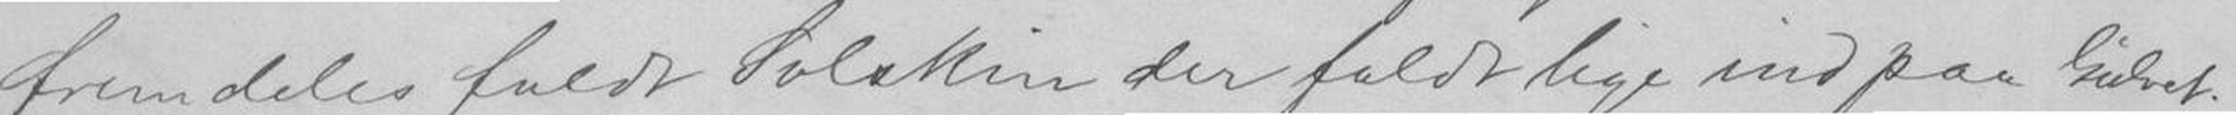fremdeles fuldt Solskin der faldt lige ind paa Gulvet
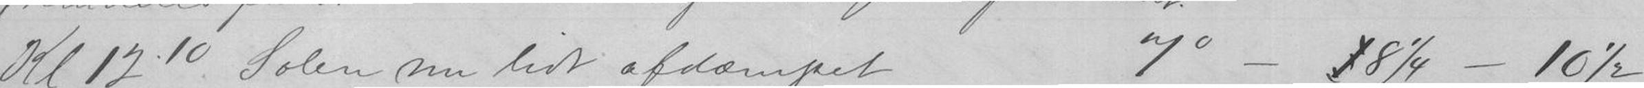Kl 12.10 Solen nu lidt afdæmpet7.- 8¼ - 10½
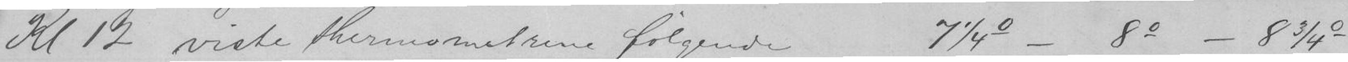Kl 12 viste thermometrene følgende7¼. - 8. - 83/4.
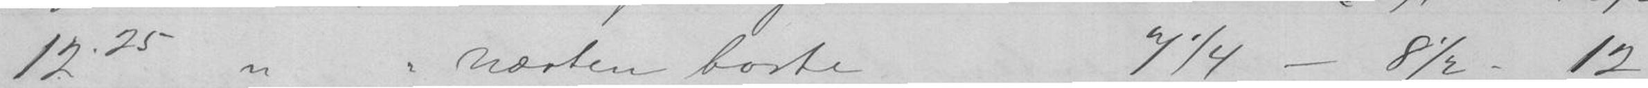" 12.25 "" næsten borte7¼- 8½ - 12
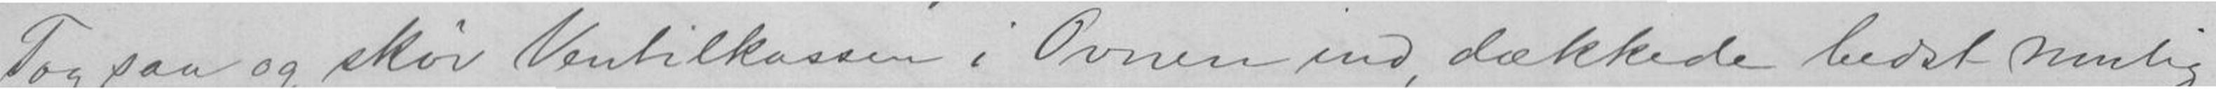Tog saa og skøv Ventilkassen i Ovnen ind, dækkede bedst mulig
